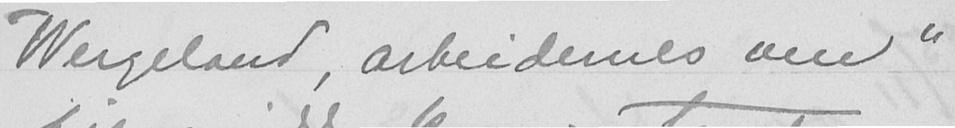Wergeland, arbeidernes ven"
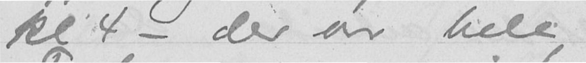kl 4 - der var hele
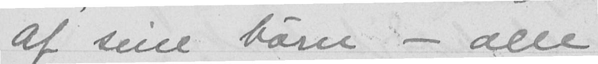af sine børn - alle
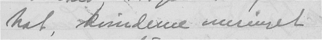hat, kvinderne omringet
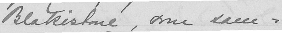Blakistone, som sam-
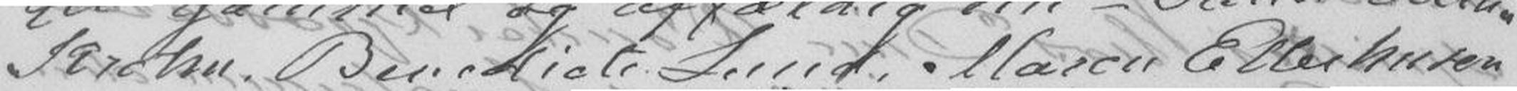Krohn. Benedicte Lund, Maren Ellerhusen
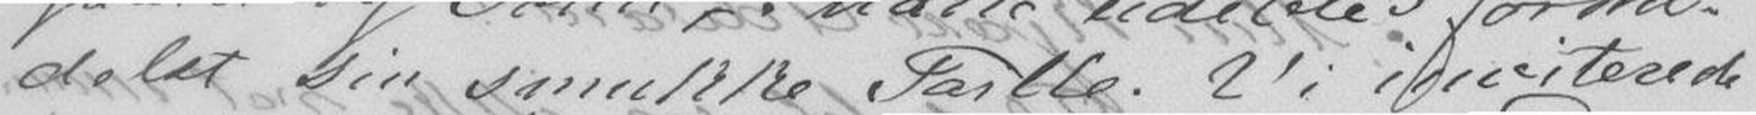dels sin smukke Tarlle. Vi inviterede
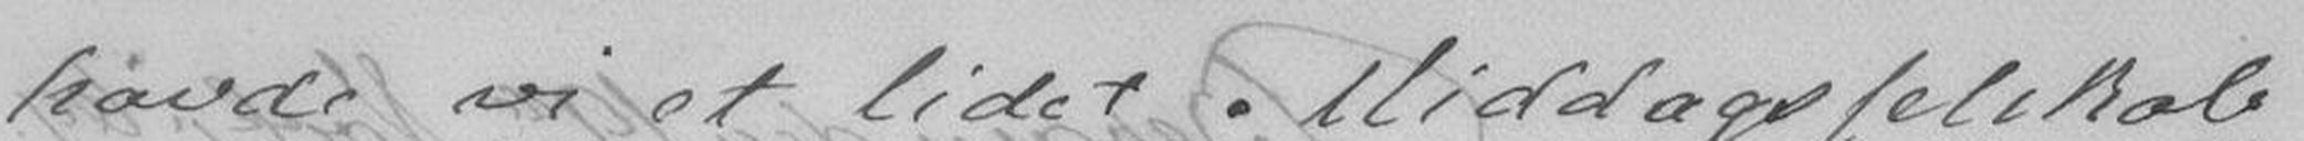havde vi et lidet Middagsselskab
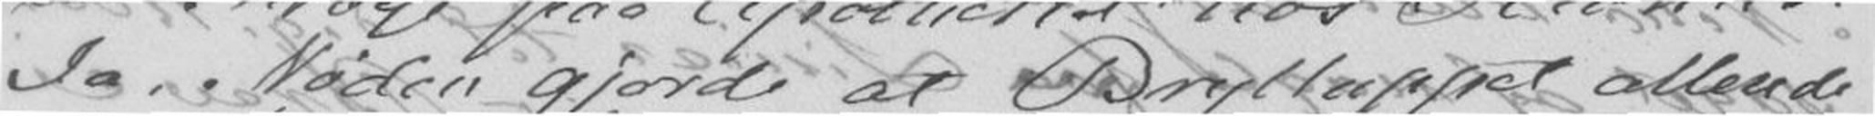Ja, Nøden gjorde at Brylluppet allerede
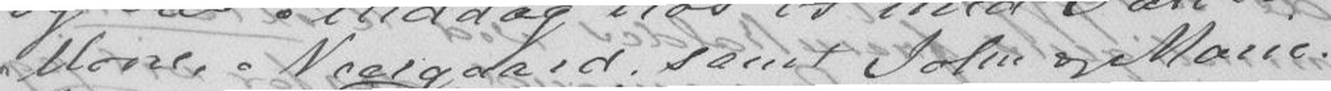Mone, Neergaard samt John og Marie.
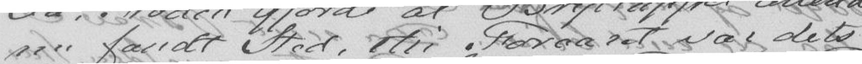nu fandt Sted, thi Foraaret var dets
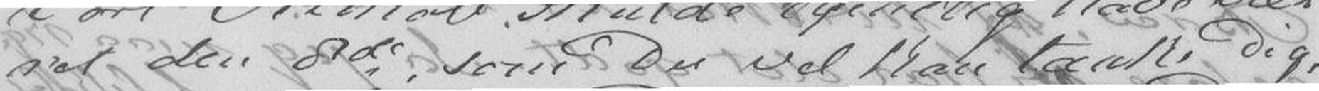ret den 8de, som Du vel kan tænke Dig
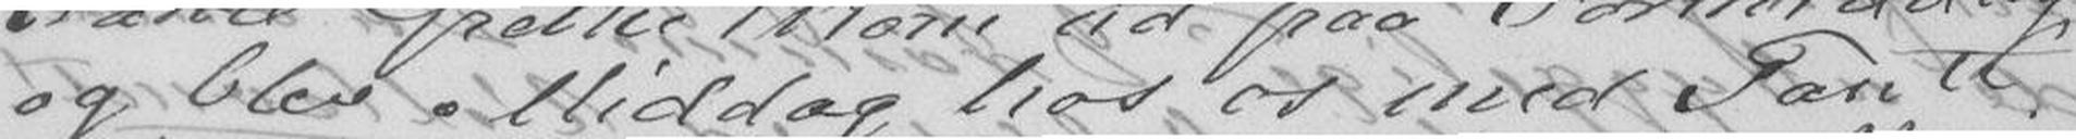og blev Middag hos os med Tante
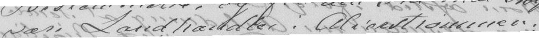være Landhandler i Alverstrømmen.
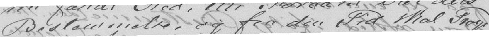Bestemmelse, og fra den Tid skal Troye
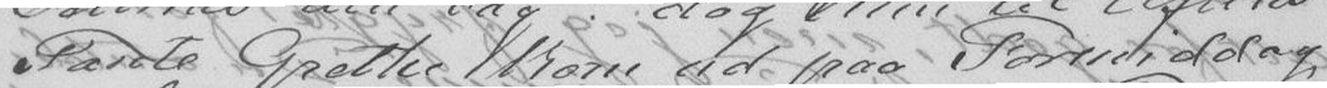Tante Grethe kom ud paa Formiddag
